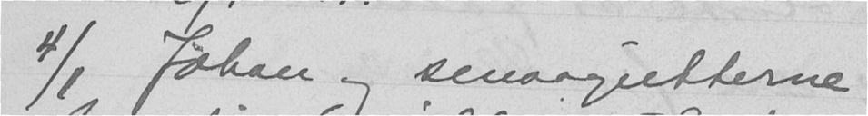4/1 Johan og smaagutterne
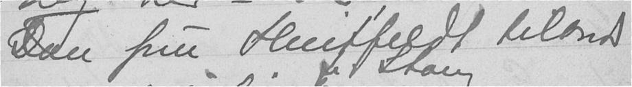Dan fru Huitfeldt tilbords
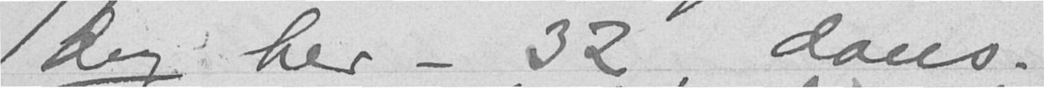Berg her - 32 dans.
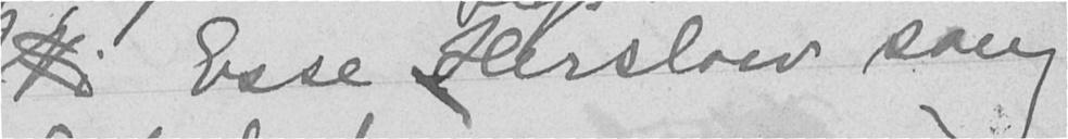Vi Esse Herslow sang
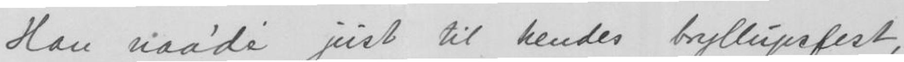Han naade just til hendes bryllupsfest,
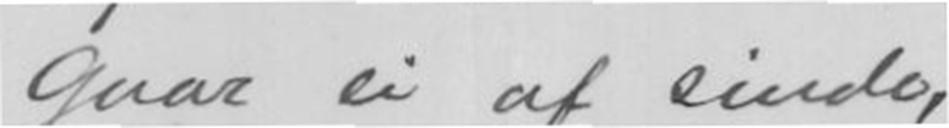Gaar ei af sinde,
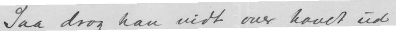Saa drog han vidt over havet ud
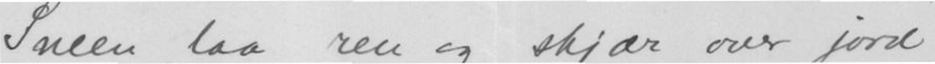Sneen laa ren og skjær over jord,
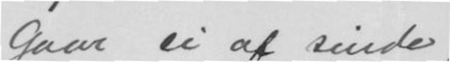Gaar ei af sinde,
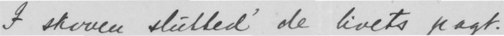I skoven slutted de livets pagt.
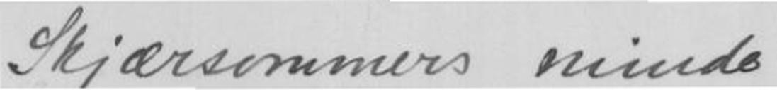Skjærsommers minde
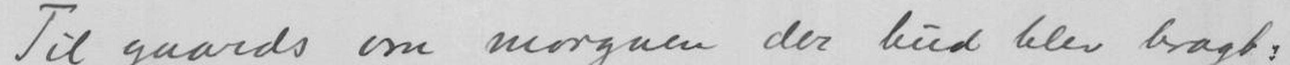Til gaards om morgenen der bud blev bragt:
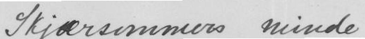Skjærsommers minde
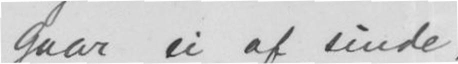gaar ei af sinde,
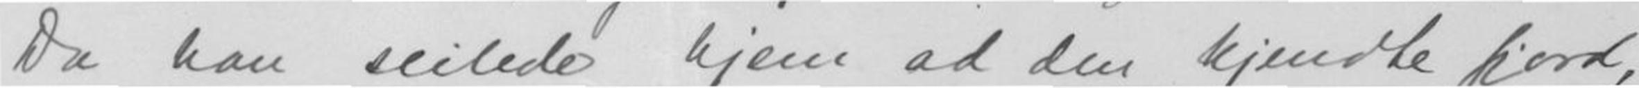Da kan seilede hjem ad den kjendte fjord,
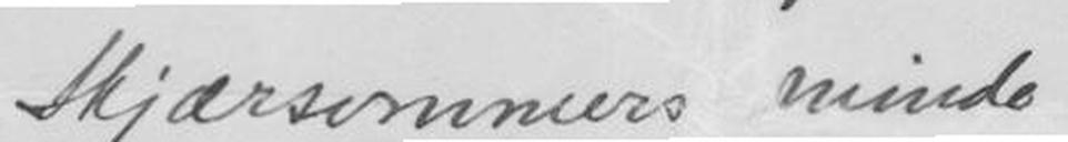Skjærsommers minde
